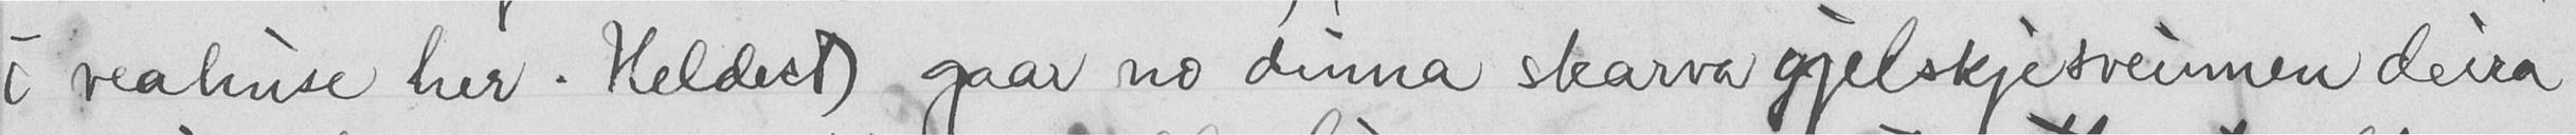i reahuse her. Heldet gaar no dinna skarva gjelskjesveimen deira
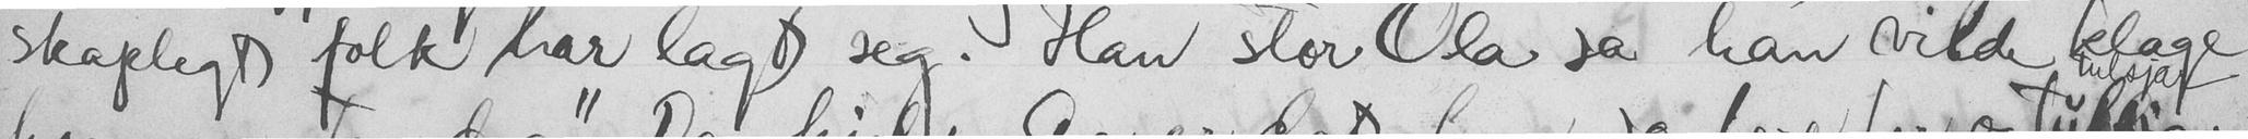skaplegt folk har lagt seg. Han stor Ola sa han vilde klage
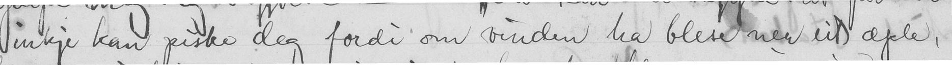inkje kan piske deg fordi om vinden ha blese ner eit æple,
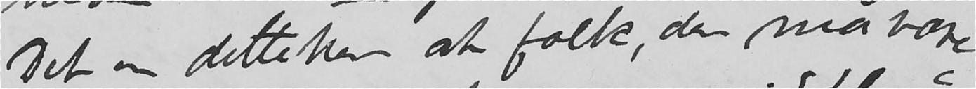Det er dette her at folk, der må være
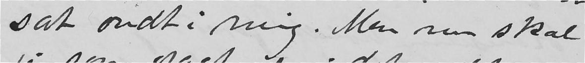sat ondt i mig. Men nu skal
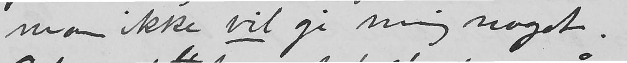man ikke vil gi mig noget.
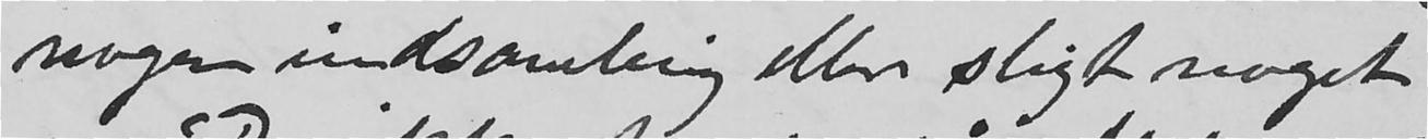nogen indsamling eller sligt noget
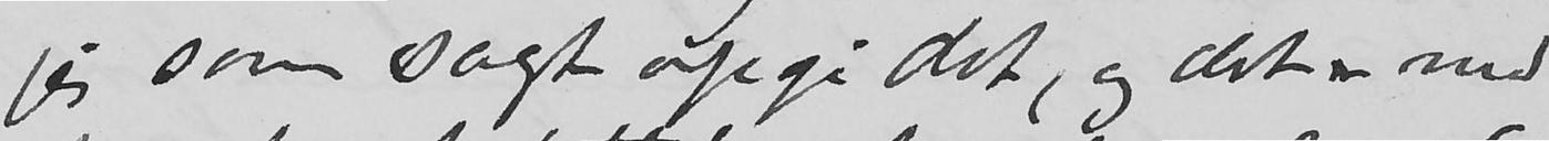jeg som sagt opgi det, og det er med
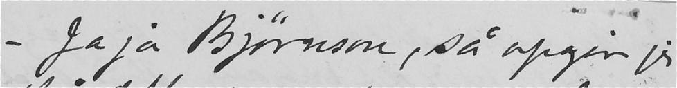- Ja ja Bjørnson, så opgir jeg
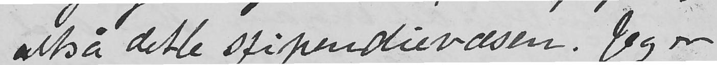altså dette stipendievæsen. Jeg er
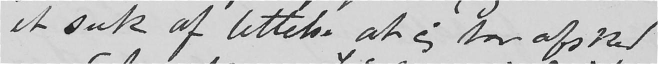et suk af lettelse at jeg tar afsked
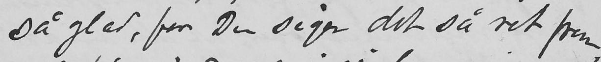så glad, for Du siger det så ret frem,
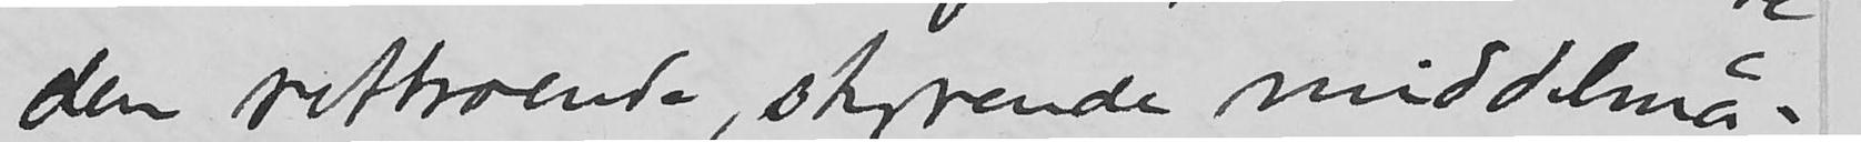den rettroende, styrende middelmå-
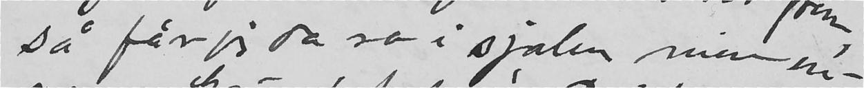så får jeg da ro i sjælen min en-
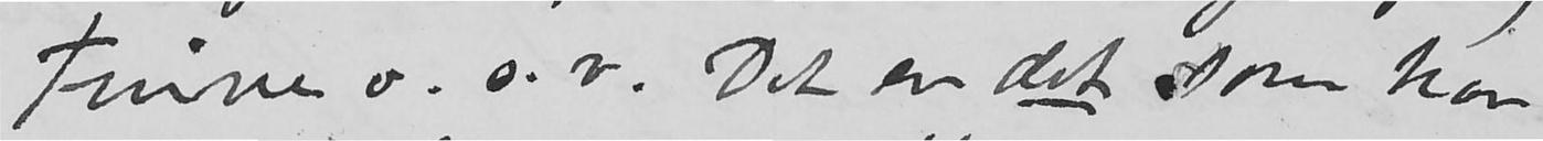Finne o. s.v. Det er det som har
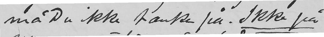må Du ikke tænke på. Ikke på
